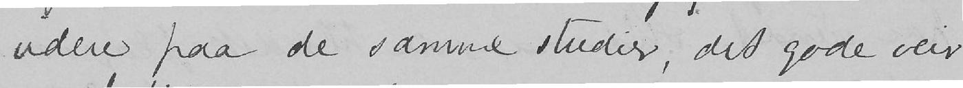videre paa de samme studier, det gode veir
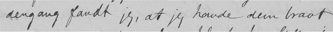dengang fandt jeg, at jeg havde dem bravt
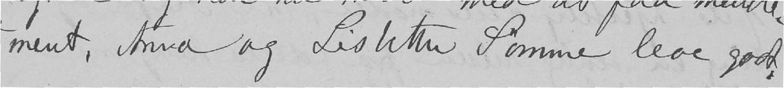ment, Anna og Lisbeth Sømme leve godt.
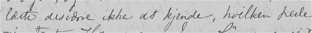lærte desværre ikke at kjende, hvilken perle
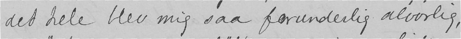det hele blev mig saa forunderlig alvorlig,
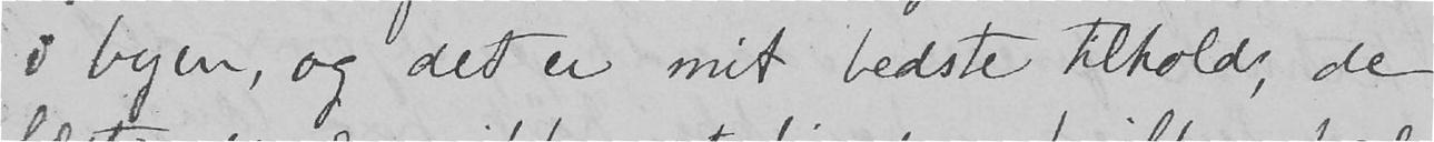i byen, og det er mit bedste tilhold, de
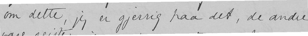om dette, jeg er gjerrig paa det, de andre
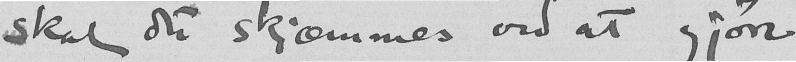skal du skjæmmes ved at gjøre
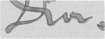Din
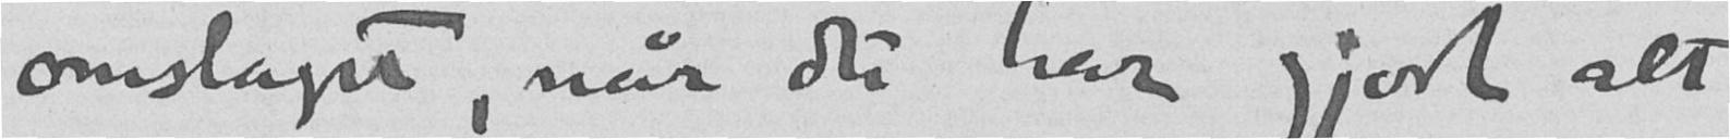omslaget, når du har gjort alt
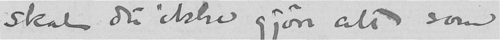skal du ikke gjøre alt som
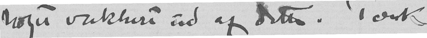noget vakkert ud af dette. Tænk
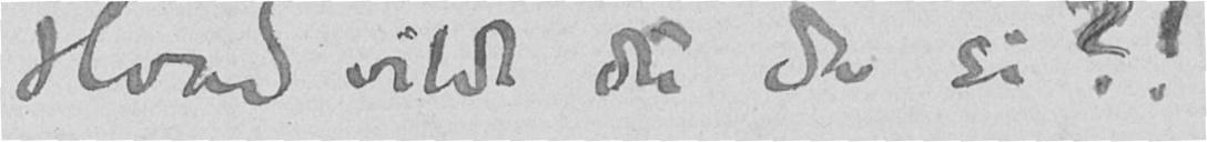Hvad vilde du da si?!
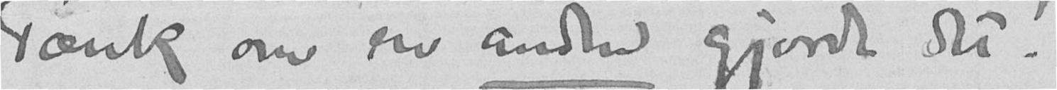Tænk om en anden gjorde det!
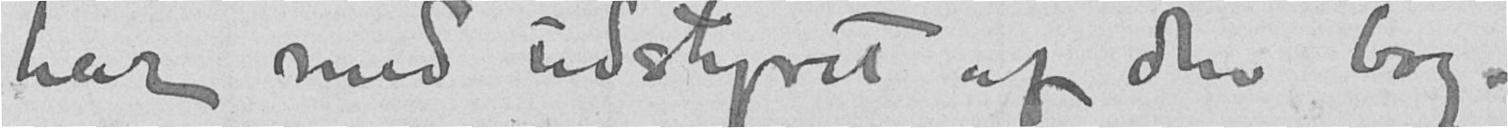har med udstyret af den bog.
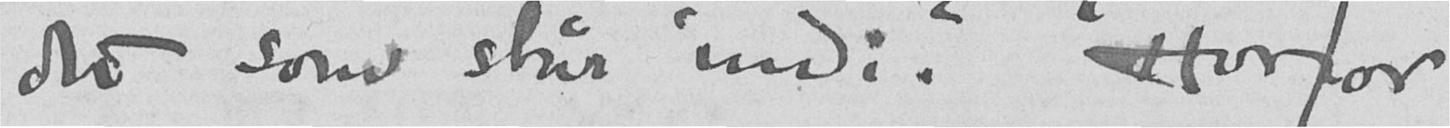det som står indi? Hvorfor
